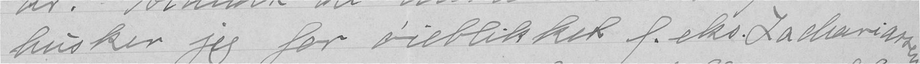husker jeg for øieblikket f.eks. Zachariassen
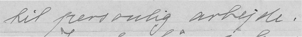til personlig arbejde.
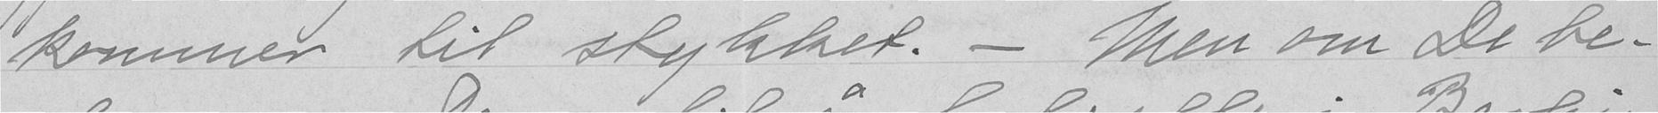kommer til stykket. - Men om De be-
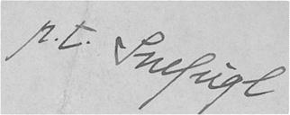p.t. Snefugl
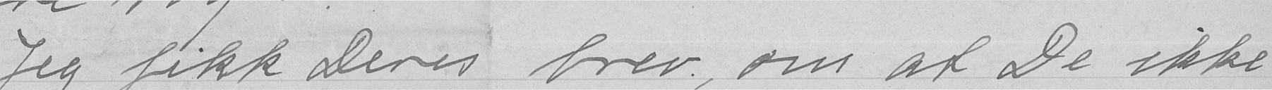Jeg fikk Deres brev, om at De ikke
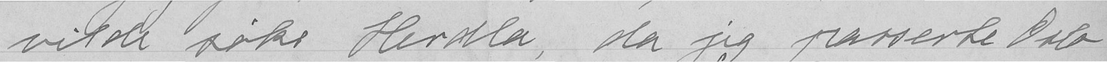vilde søke Herdla, da jeg passerte Oslo
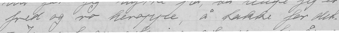fred og ro heroppe, å takke for det.
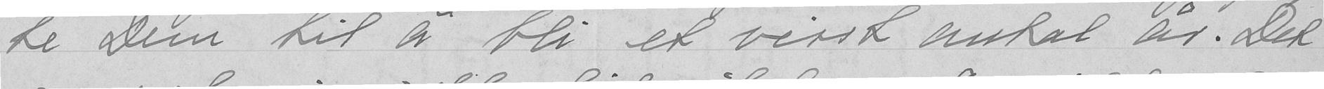te Dem til å bli et visst antal år. Det
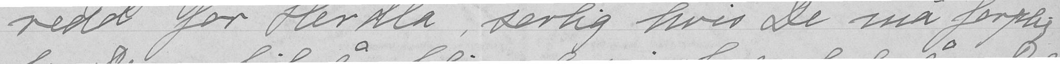redd for Herdla, særlig hvis De må forplig-
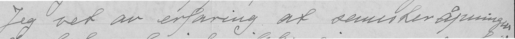Jeg ved av erfaring at semesteråpningen
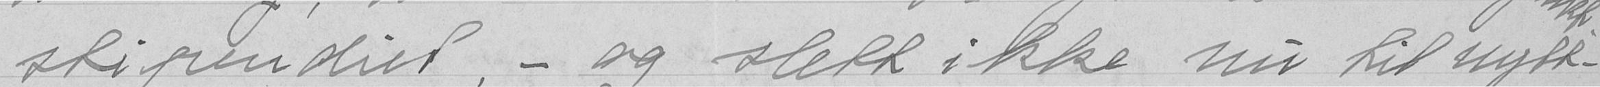stipendiet, - og slett ikke nu til nytt-
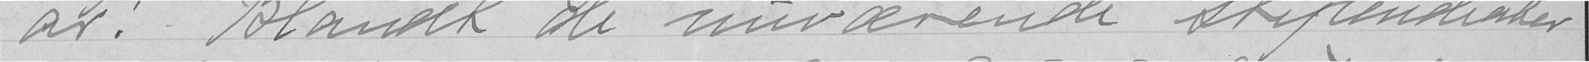år. Blandt de nuværende stipendiater
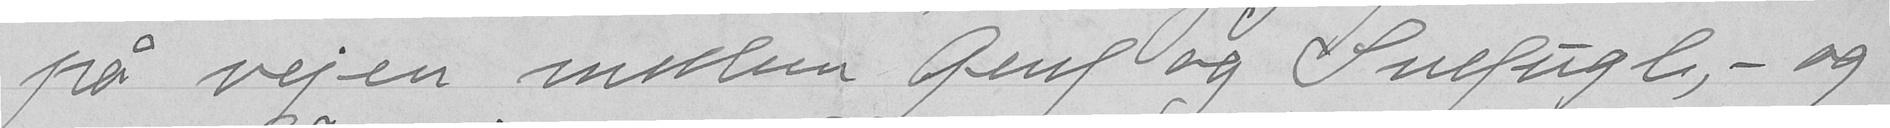på vejen mellem Gent og Snefugl, - og
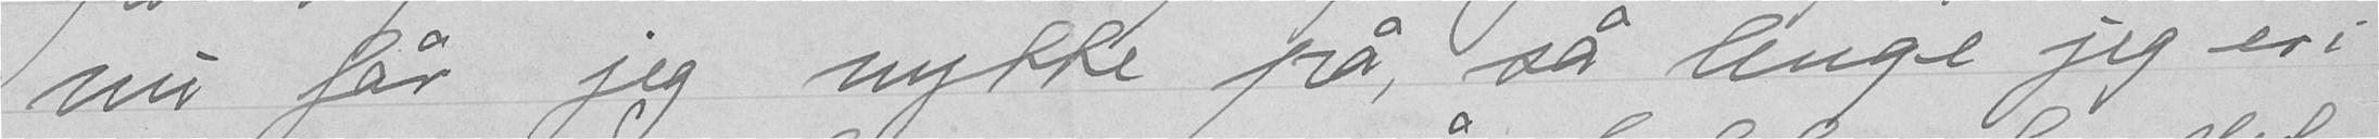nu får jeg nytte på, så lenge jeg er i
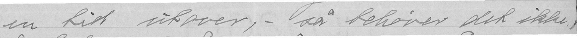en til utover, - så behøver det ikke
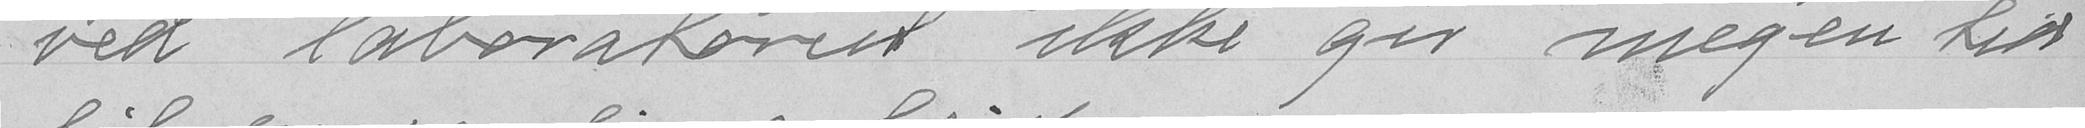ved laboratoriet ikke gir megen tid
